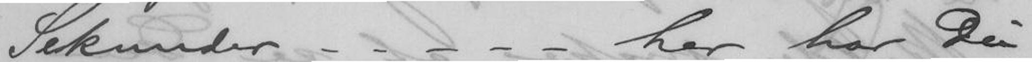Sekunder - - - - - her har Du
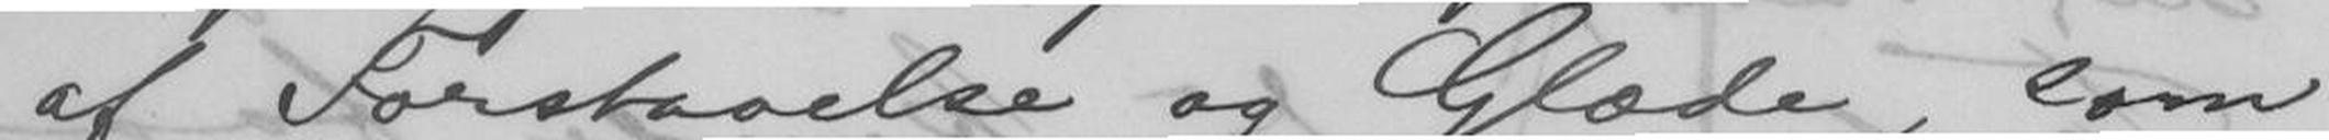af Forstaaelse og Glæde, som
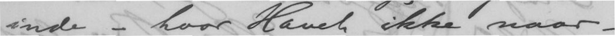inde - hvor Havet ikke naar -
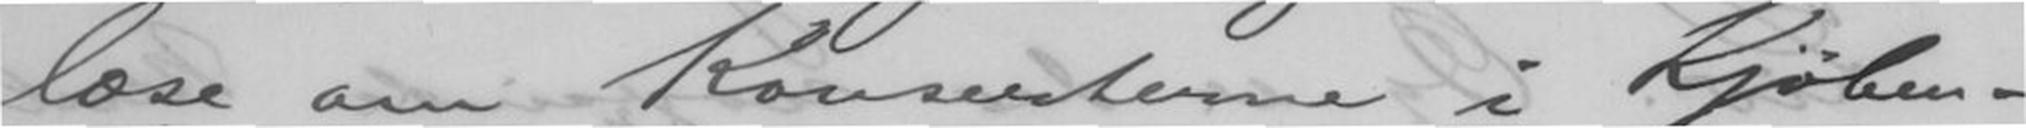læse om Konserterne i Kjøben-
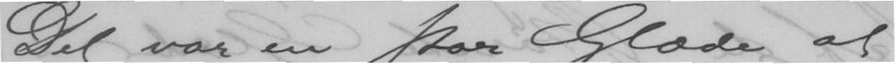Det var en stor Glæde at
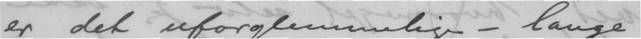er det uforglemmelige - lange -
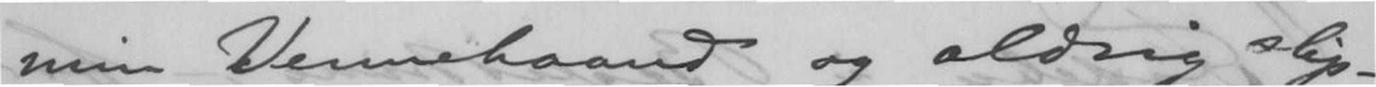min Vennehaand og aldrig slip-
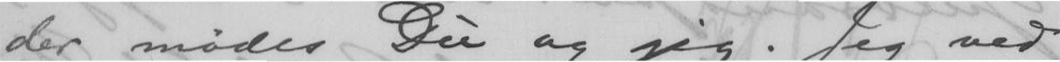der mødes Du og jeg. Jeg ved
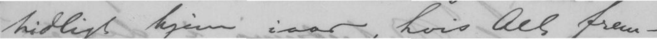tidligt hjem iaar, hvis Alt frem-
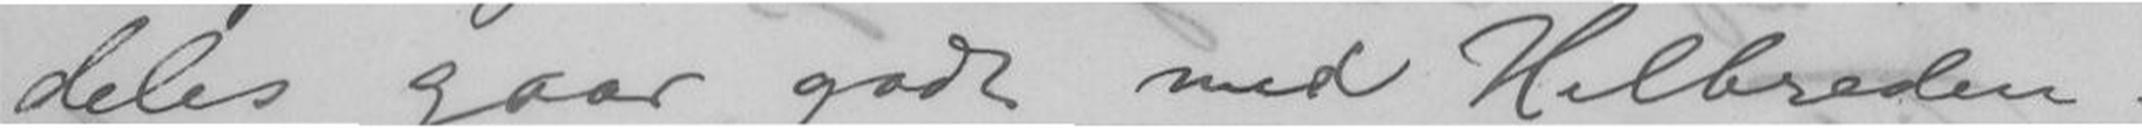deles gaar godt med Helbreden.
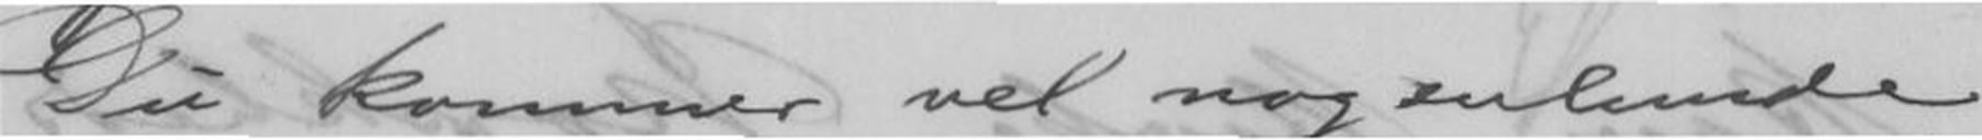- Du kommer vel nogenlunde
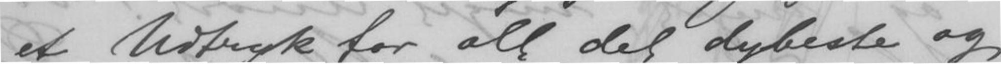et Udtryk for alt det dybeste og
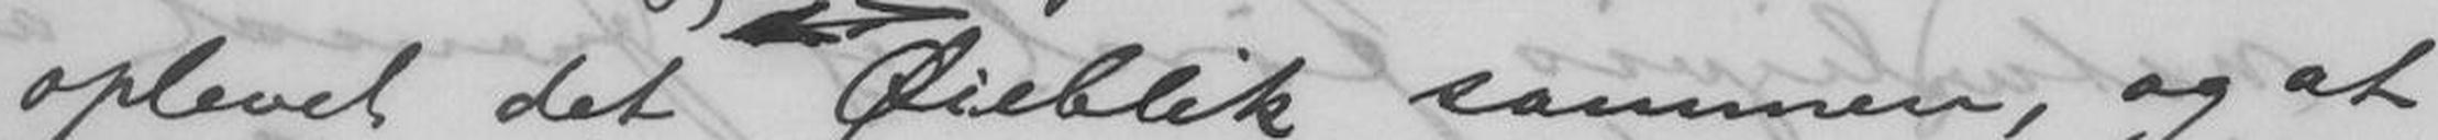oplevet det Øieblik sammen, og at
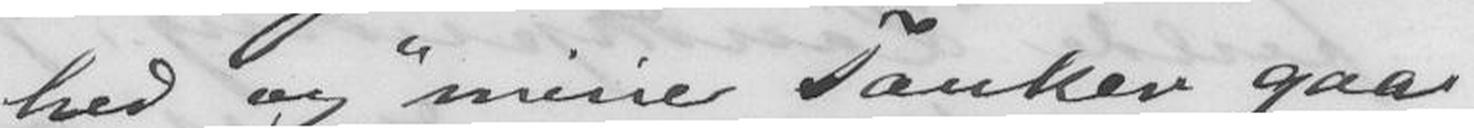hed og "mine Tanker gaar
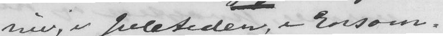nu, i Juletiden, i Ensom-
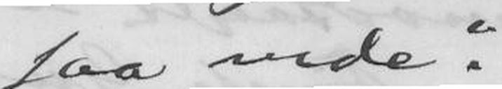saa vide."
